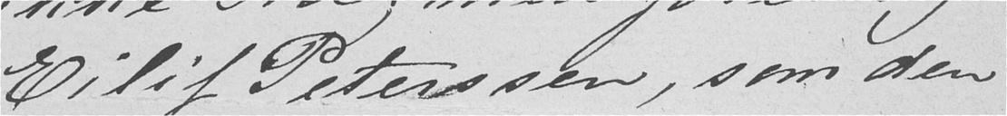Eilif Peterssen, som den
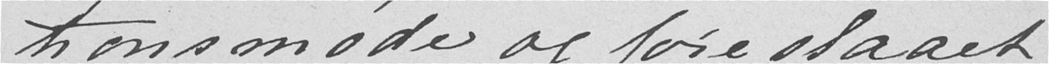tionsmøde og foreslaaet,
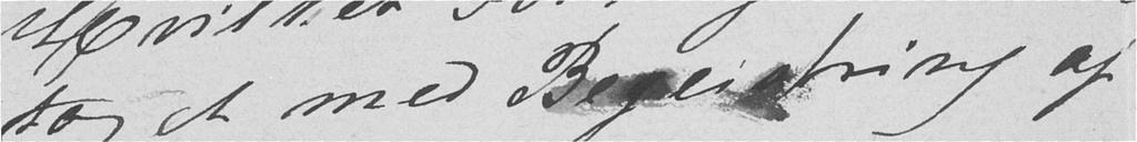taget med Begeistring af
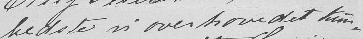bedste vi overhovedet kun-
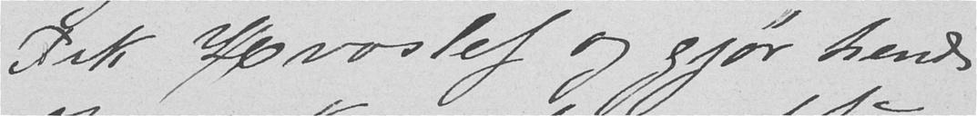Frk Hvoslef og gjør hende
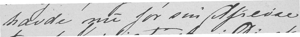havde nu før sin Afreise
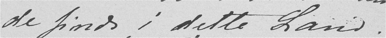de finde i dette Land.
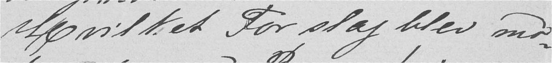Hvilket Forslag blev mod-
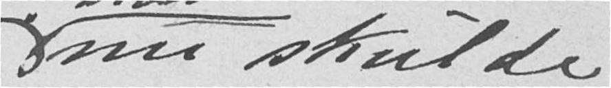nu skulde
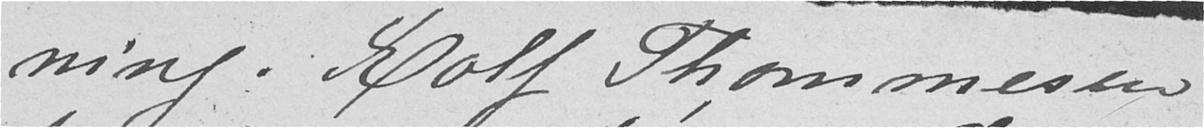ning. Rolf Thommesen
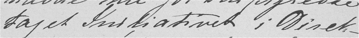taget Indtiativet i Direk-
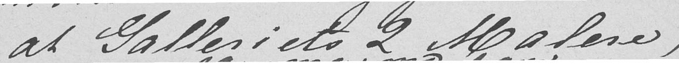at Galleriets 2 Malere,
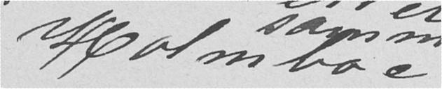Holmboe og jeg
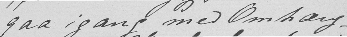gaa igang med Omhæng-
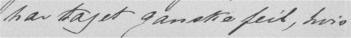har taget ganske feil, hvis
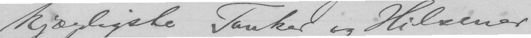kjærligste Tanker og Hilsener
